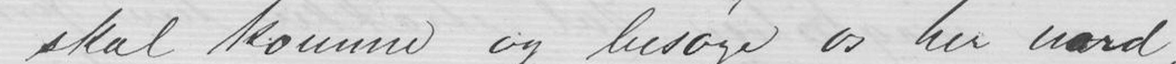skal komme og besøge os her nord,
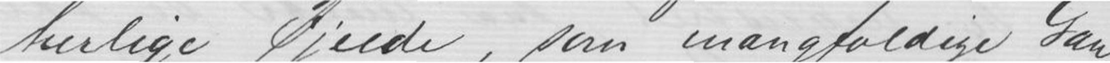herlige Fjelde, som mangfoldige Gan-
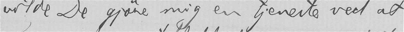vilde De gjøre mig en tjeneste ved at
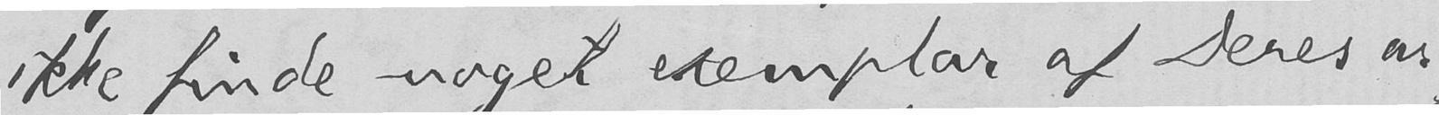ikke finde noget exemplar af Deres ar-
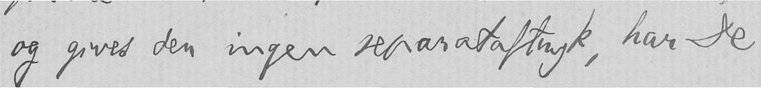og gives der ingen separataftryk, har De
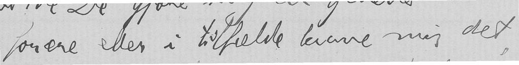forære eller i tilfælde laane mig det,
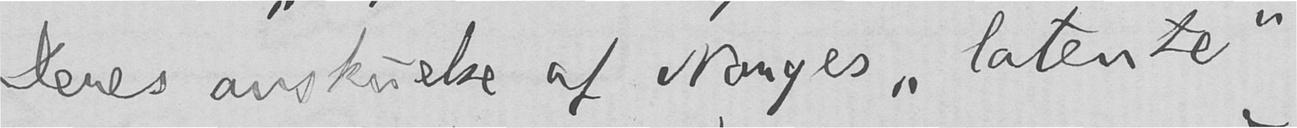Deres anskuelse af Norges "latente"
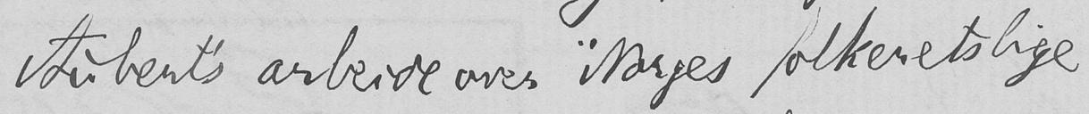Aubert's arbeide over "Norges folkeretslige
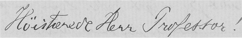Høistærede Herr Professor!
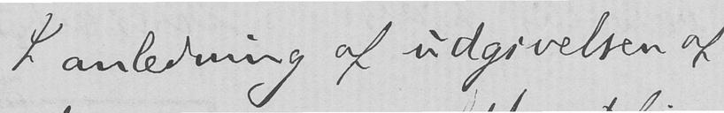I anledning af udgivelsen af
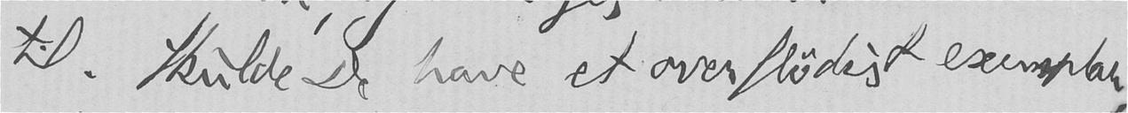til. Skulde De have et overflødigt exemplar,
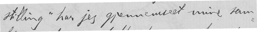stilling" har jeg gjennemseet mine sam-
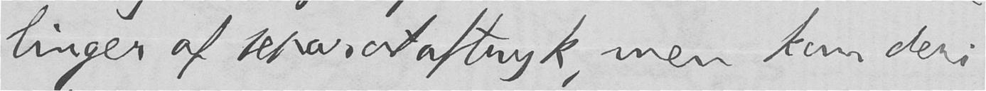linger af separataftryk, men kan deri
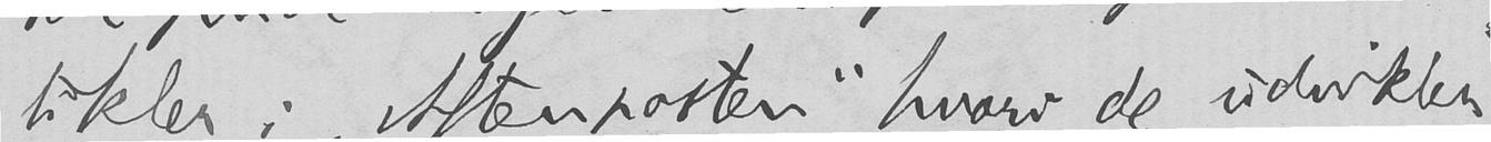tikler i "Aftenposten", hvori de udvikler
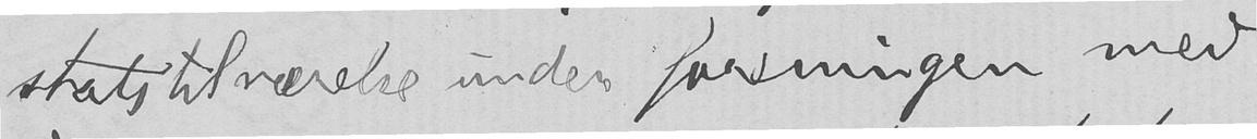statstilværelse under foreningen med
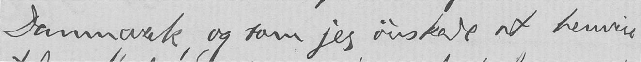Danmark, og som jeg ønskede at henvise
